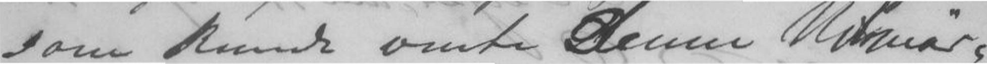som kunde vente Denne Udmär-
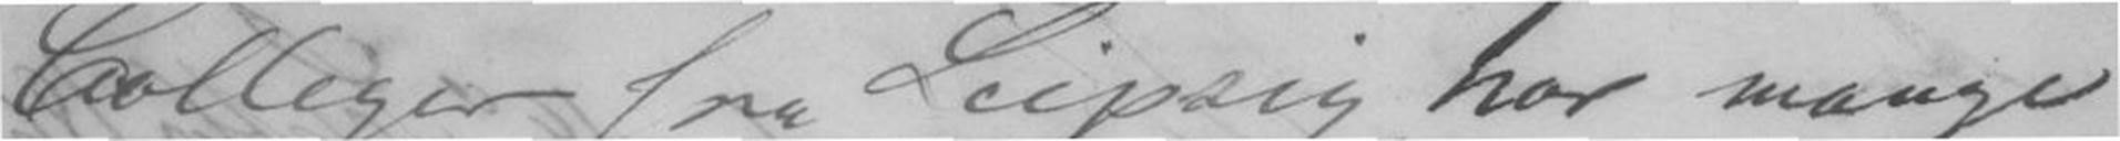Colleger fra Leipzig har mange
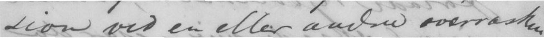sion ved en eller anden overrasken-
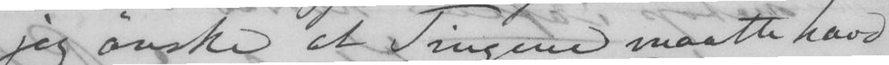jeg ønske at Tingene maatte havg
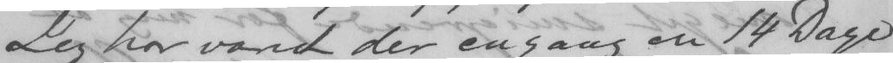Jeg har været der engang om 14 Dage
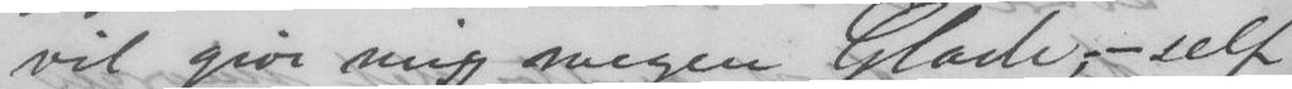vil give mig megen Glæde; - self
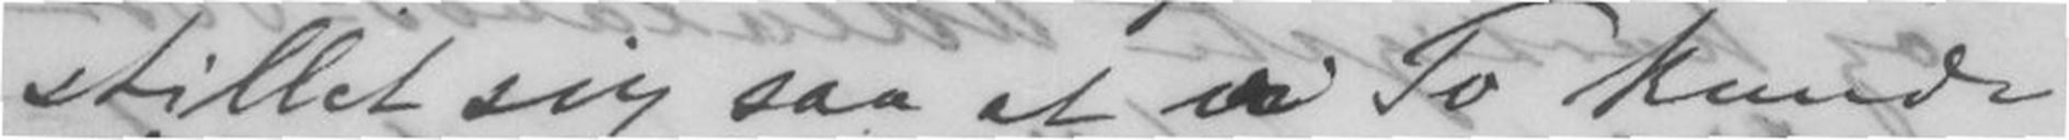stillet sig saa at vi To kunde
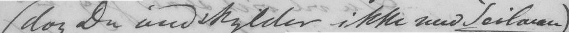(dog Du undskylder ikke med Teiloven)
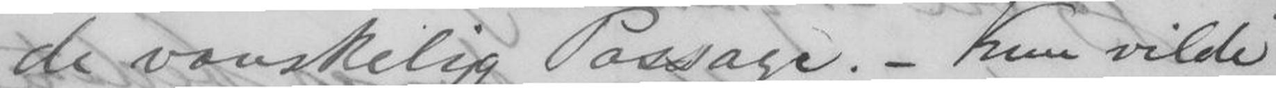de vanskelig Passage. - Kun vilde
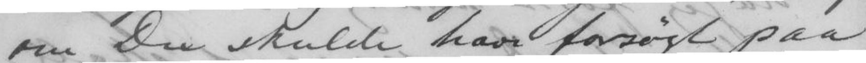on Du dkulde have forsøgt paa
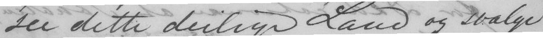see dette deilige Land og svælge
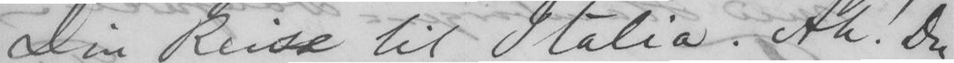Din Reise til Italien. Ah! Du
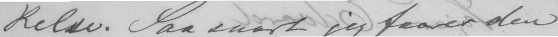kelse. Saa snart jeg faaer den
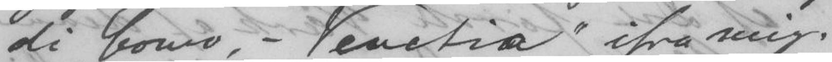di Cowo, - Venetia" ifra mig.
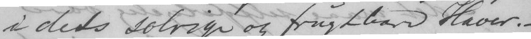i dets solrige og frugtbare Haver. -
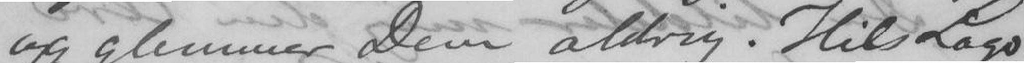og glemmer Dem aldrig. Hils Lago
